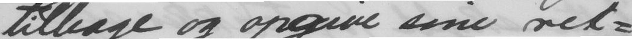tilbage og opgive sine ret-
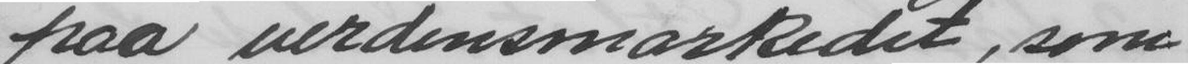paa verdensmarkedet, som
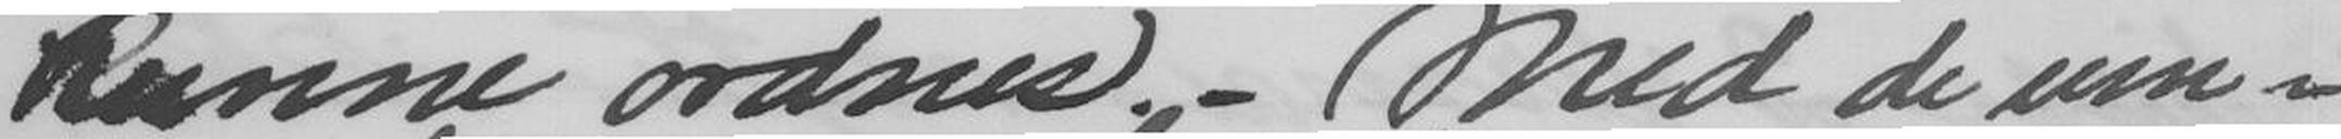kunne ordnes. - Med de ven-
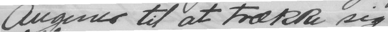Augener til at trække sig
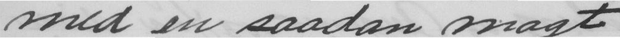med en saadan magt
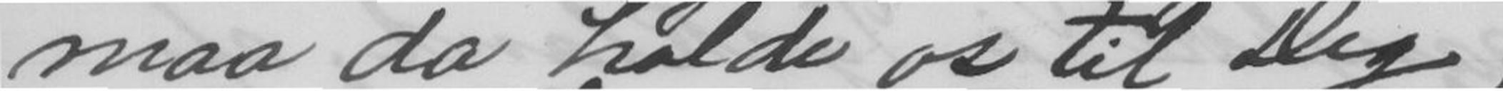maa da holde os til Dig,
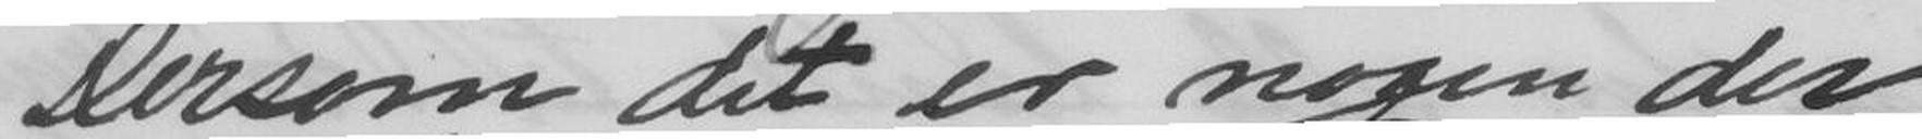Dersom det er nogen der
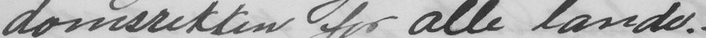domsretten for alle lande. -
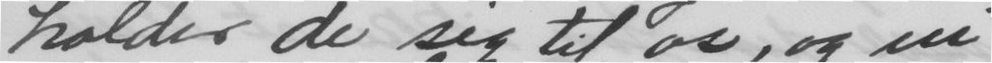holder de sig til os, og vi
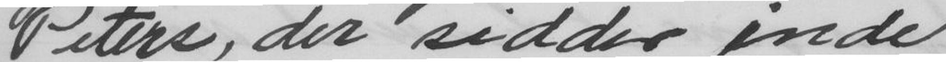Peters, der sidder inde
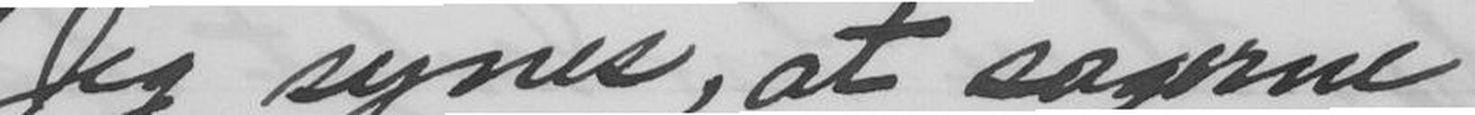Jeg synes, at sagerne
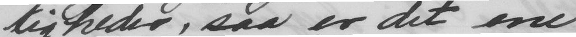tigheder, saa er det ene
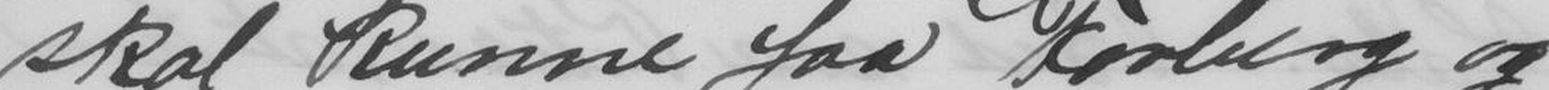skal kunne faa Forberg og
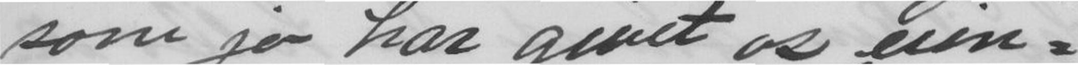som jo har givet os eien-
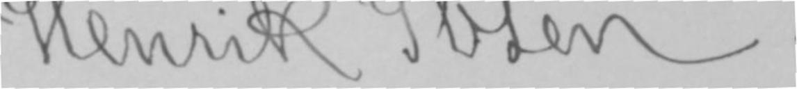Henrik Ibsen.
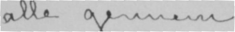alle gennem
